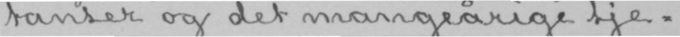tanter og det mangeårige tje-
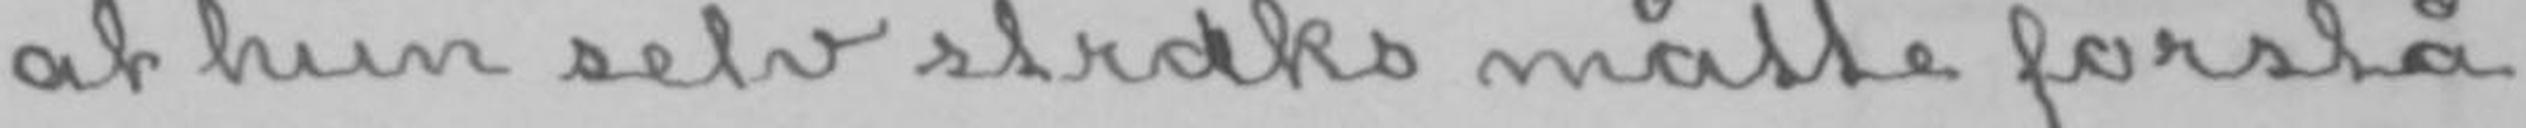at hun selv straks måtte forstå
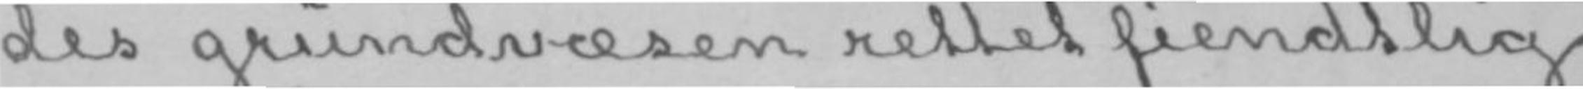des grundvæsen rettet fiendtlig
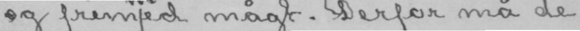og fremmed mågt. Derfor må de
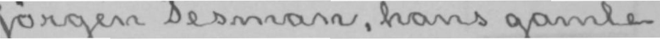Jørgen Tesman, hans gamle
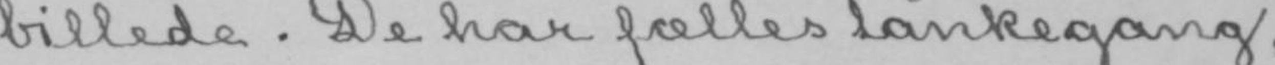billede. De har fælles tankegang,
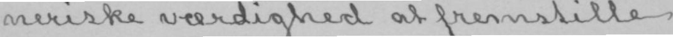neriske værdighed at fremstille
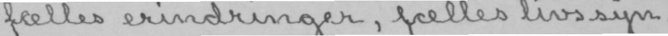fælles erindringer, fælles livssyn.
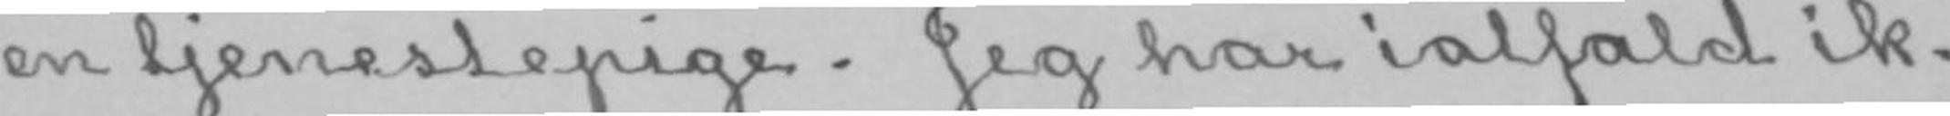en tjenestepige. Jeg har ialfald ik-
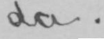da.
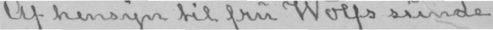Af hensyn til fru Wolfs sunde
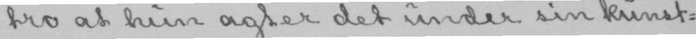tro at hun agter det under sin kunst-
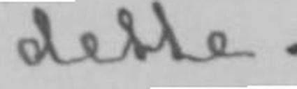dette.
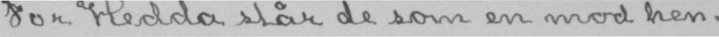For Hedda står de som en mod hen-
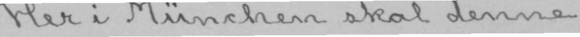Her i München skal denne
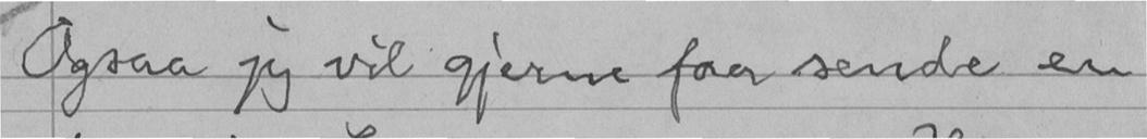Ogsaa jeg vil gjerne faa sende en
